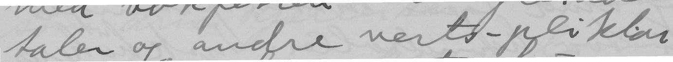taler og andre verts-pliktar
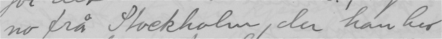no frå Stockholm, der han hev
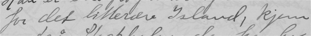for det litterære Island, kjem
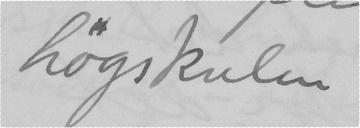högskulen
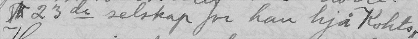23de selskap for han hjå Kohts,
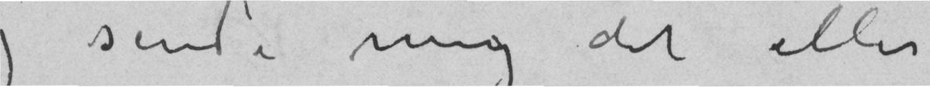og sende mig det eller
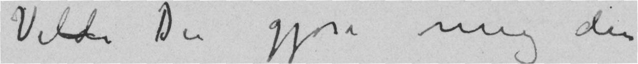Vilde Du gjøre mig den
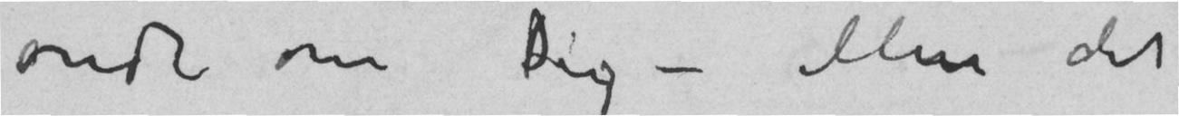ondt om Dig – Men det
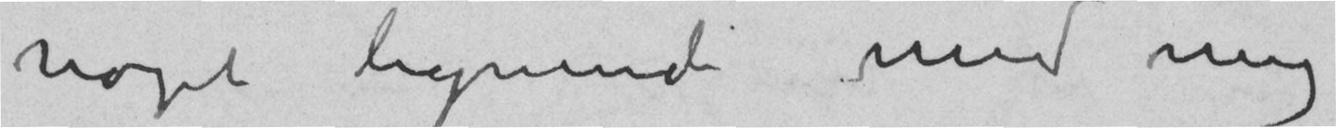noget lignende med mig
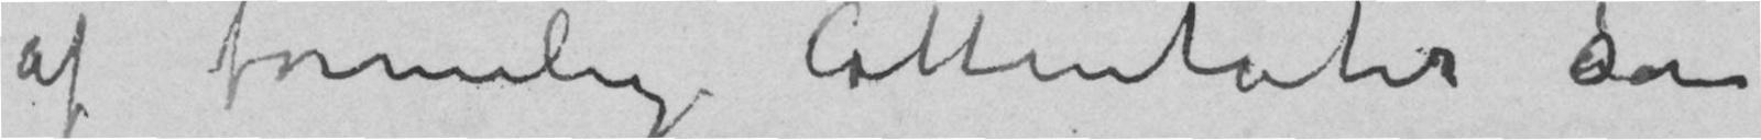af formelig Attentater som
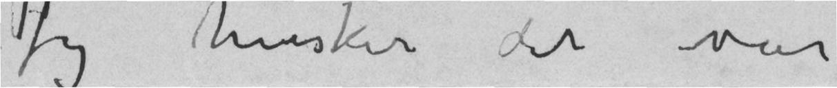Jeg husker det var
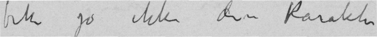fik jo ikke den Karakter
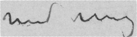med mig
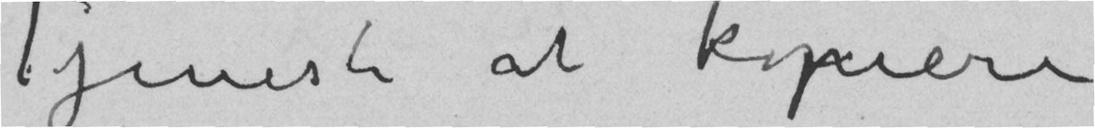Tjeneste at kopiere
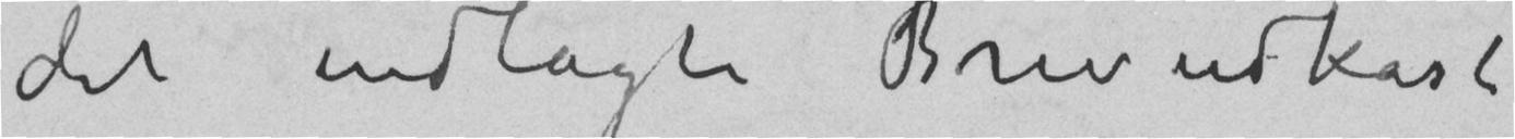det indlagte Brevudkast
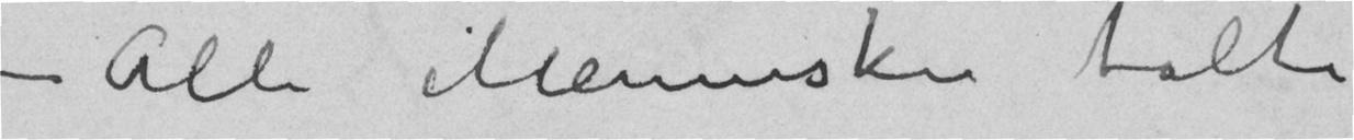– Alle Mennesker talte
